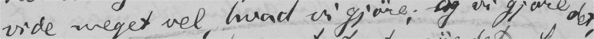vide meget vel, hvad vi gjøre; og vi gjøre det,
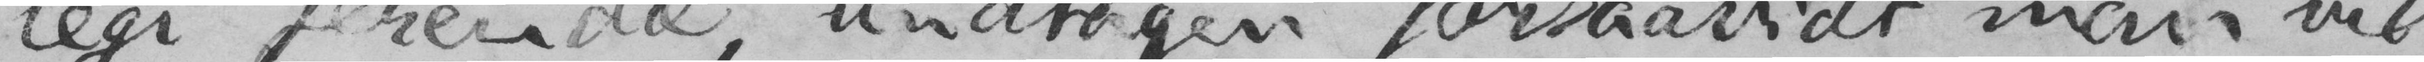legi ferendæ, undtagen forsaavidt man vil
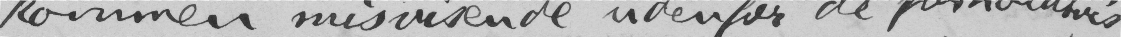kommen misvisende udenfor de forholdsvis
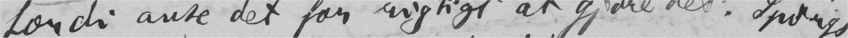fordi anse det for rigtigt at gjøre det. Spørgs-
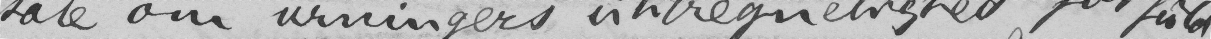tale om urningers utilregnelighed for fuld-
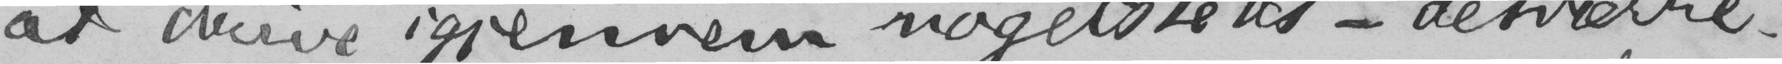at drive igjennem nogetsteds – desværre.
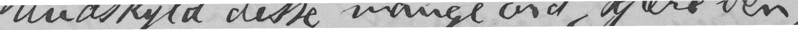Undskyld disse mange ord, kjære ven;
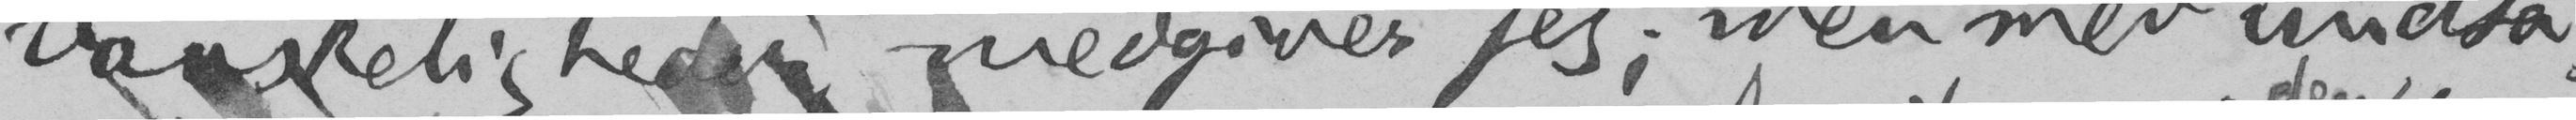vanskeligheder medgiver jeg; men med undta-
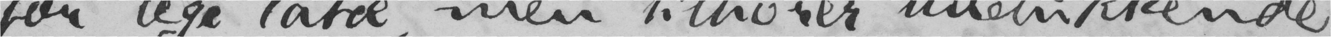for legi latæ, men tilhører udelukkende
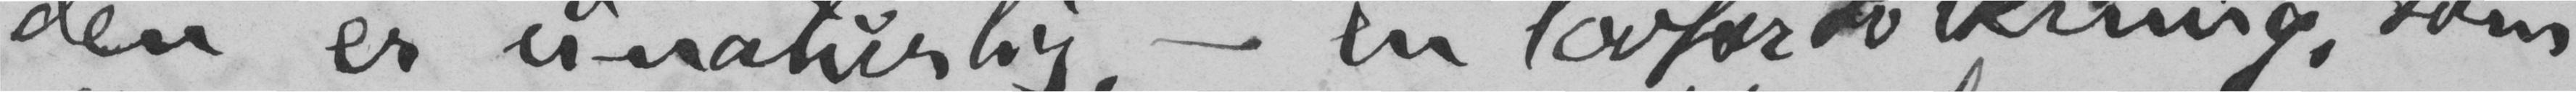den er unaturlig, - en lovfortolkning, som
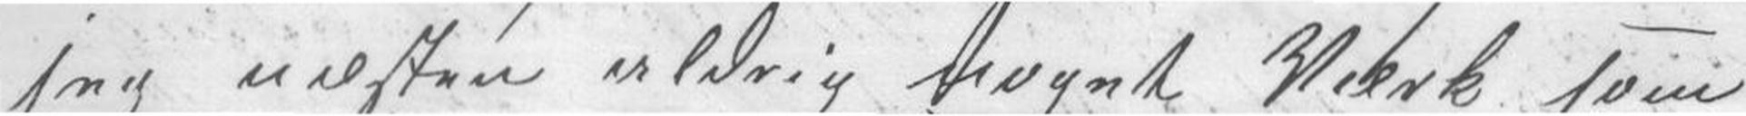jeg næsten aldrig noget Værk som
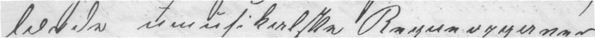abelser umusikalske Regneopgaver.
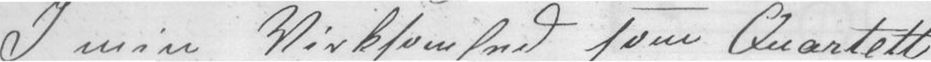I min Virksomhed som Quartett-
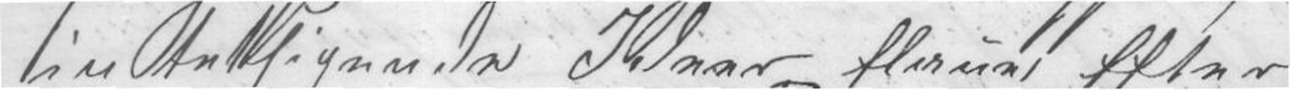intetsigende Ideer flaue Efter-
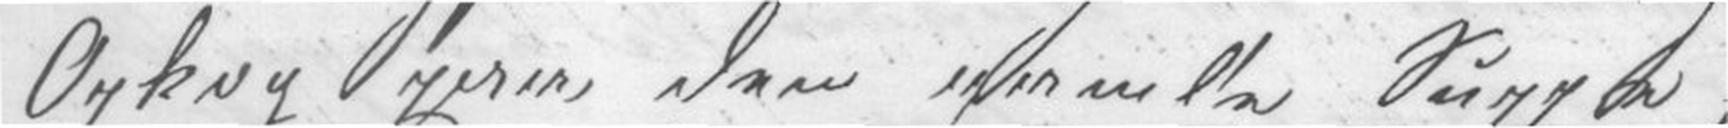Opkog paa den gamle Suppa,
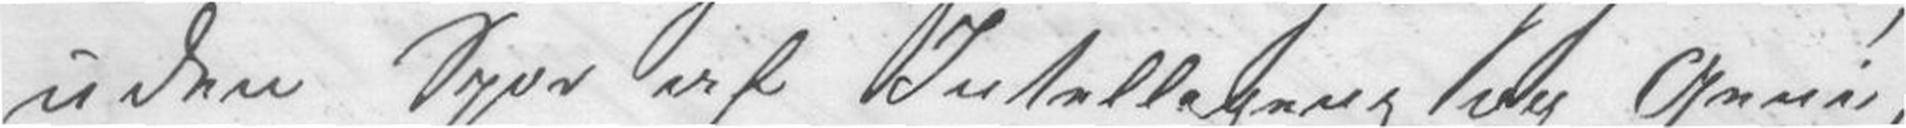uden Syn af Intellegenz og Geni,
